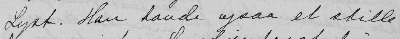Lyst. Han havde ogsaa et stille
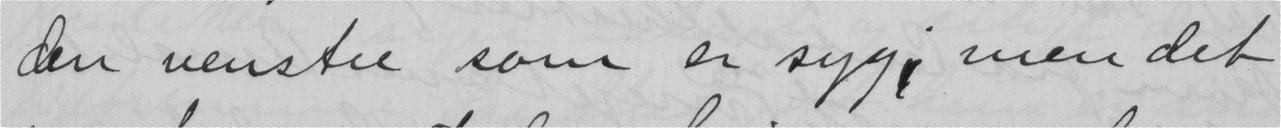den venstre som er syg; men det
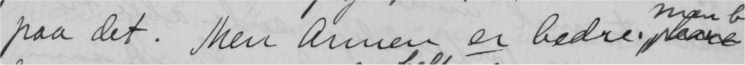paa det. Men armen er bedre,
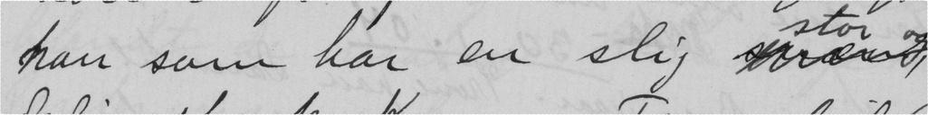han som har en slig
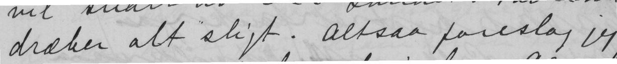dræber alt sligt. Altsaa foreslog jeg
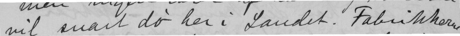vil snart dø her i Landet. Fabrikkerne
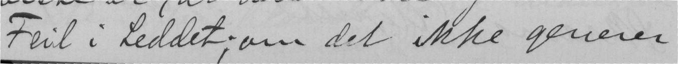Feil i Leddet; om det ikke generer
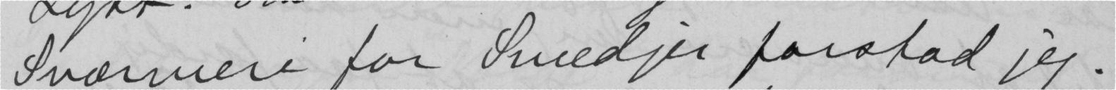Sværmeri for Smedjer forstod jeg.
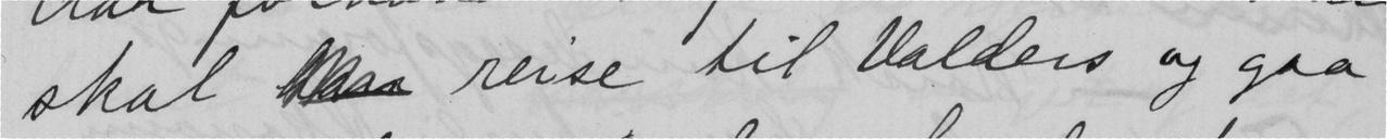skal  reise til Valders og gaa
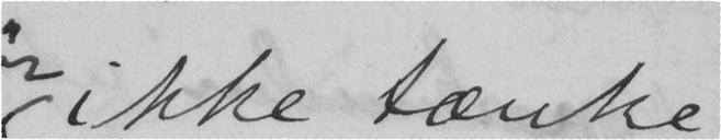ikke tænke
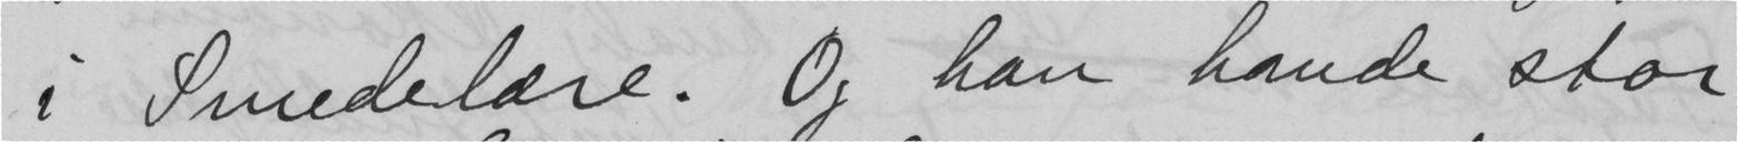i Smedelære. Og han havde stor
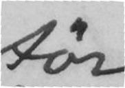tør
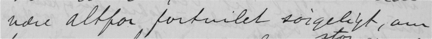være altfor fortvilet sørgeligt, om
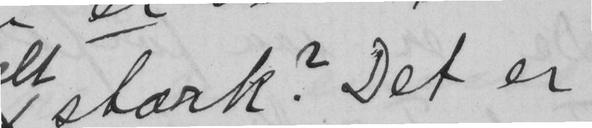stærk? Det er
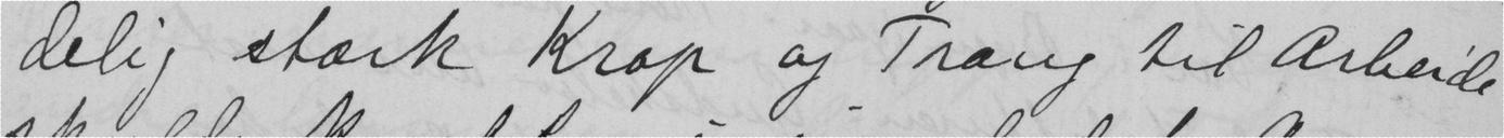delig stærk Krop og Trang til arbeide
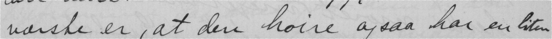værste er, at den høire ogsaa har en liten
